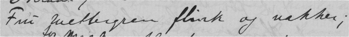Fru Wettergren flink og vakker;
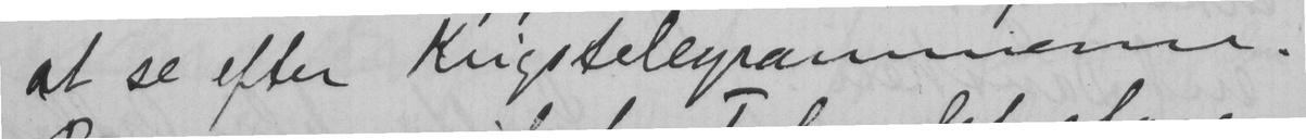at se efter Krgstelegrammerne.
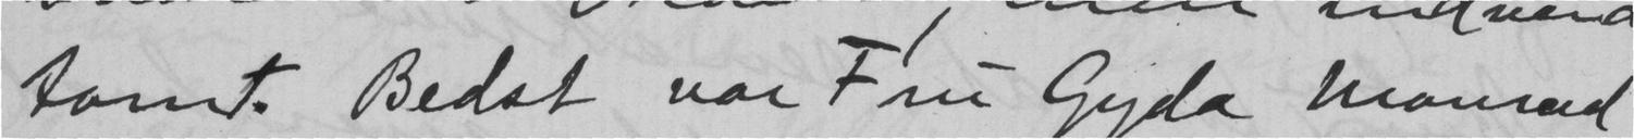tomt. Bedst var Fru Gyda Monrad
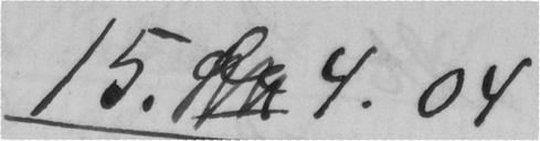15. 4.04
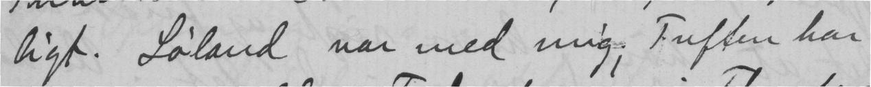ligt. Løland var med mig; Tuften har
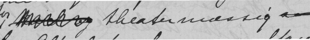theatermæssig
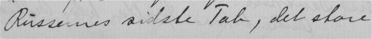Russernes sidste Tab, det store
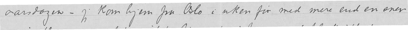aarsdagen – jeg kom hjem fra Oslo i uken før med mere end en snev
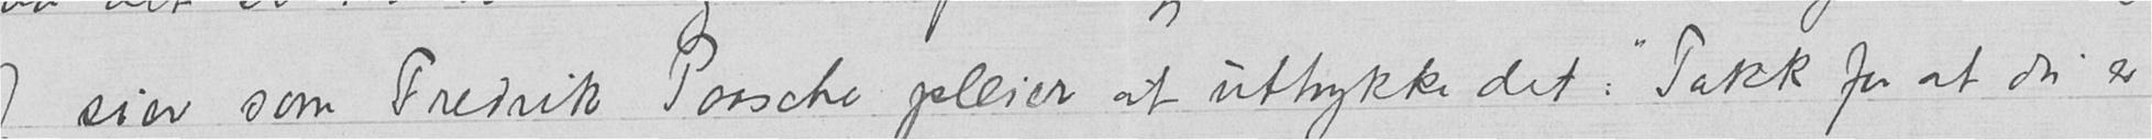Jeg sier som Fredrik Paasche pleier at uttrykke det: "Takk for at du er
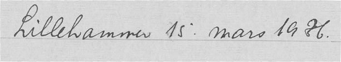Lillehammer 15. mars 1936.
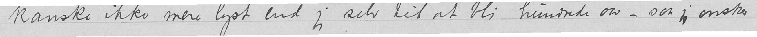kanske ikke mere lyst end jeg selv til at bli hundrede aar – saa jeg ønsker
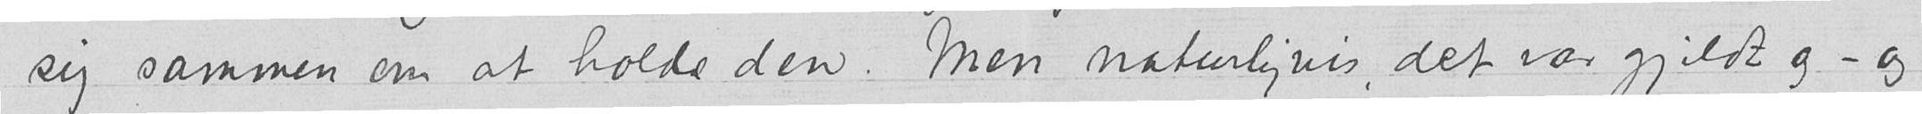sig sammen om at holde den. Men naturligvis, det var gjildt og – og
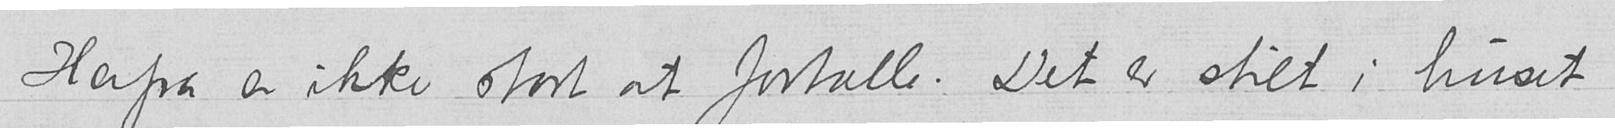Herfra er ikke stort at fortælle. Det er stilt i huset
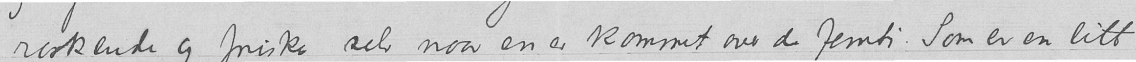raskende og friske, selv naar en er kommet over de femti. Som er en litt
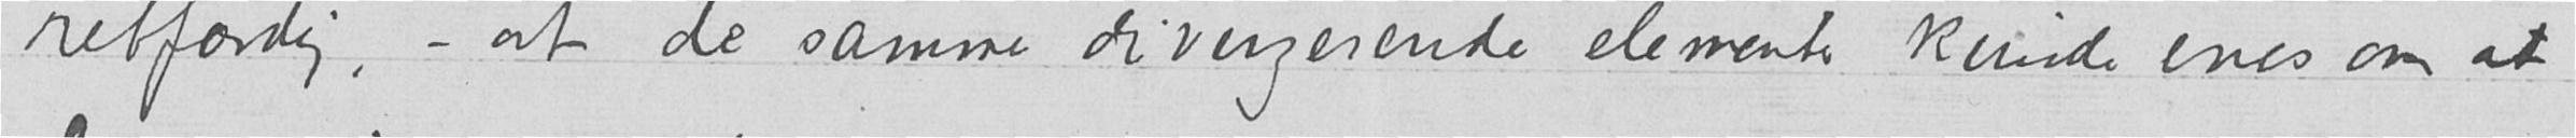retfærdig, – at de samme divergerende elementer kunde enes om at
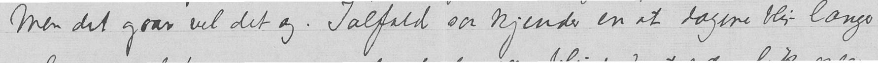Men det gaar vel det og. Ialfald saa kjender en at dagene blir længer
